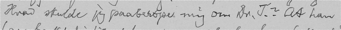Hvad skulde jeg paaberope mig om Dr. T.? At han
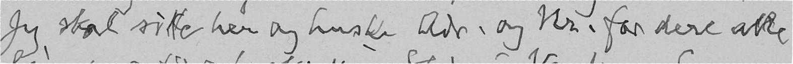Jeg skal sitte her og huske Adr. og Nr. for dere alle
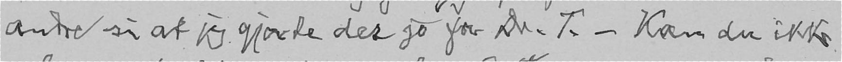andre si at jeg gjorde det jo for Dr. T. - Kan du ikke
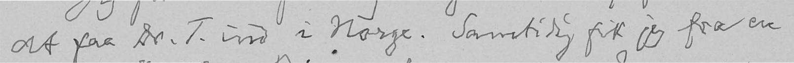at faa Dr. T. ind i Norge. Samtidig fik jeg fra en
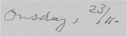Onsdag, 23/11.
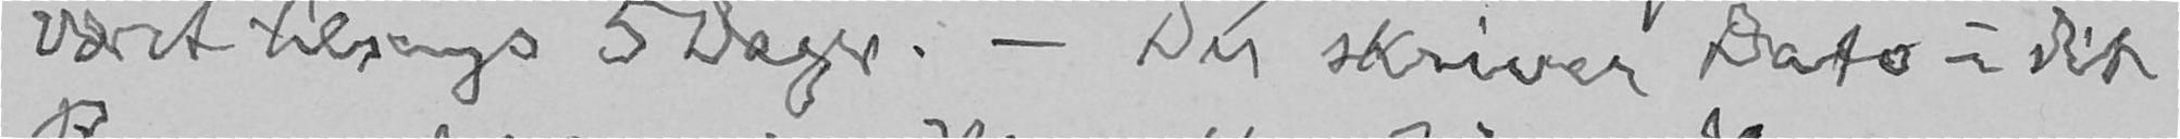været tilsengs 5 Dager. - Du skriver Dato i dit
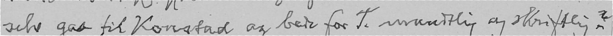selv gaa til Konstad og bede for T. mundtlig og skriftlig?
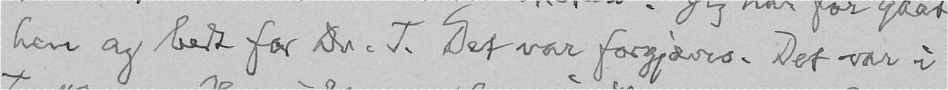hen og bedt for Dr. T. Det var forgjæves. Det var i
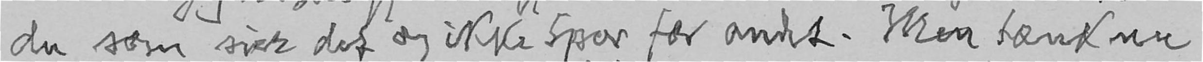du som sier det og ikke Spor for andet. Men tænk nu
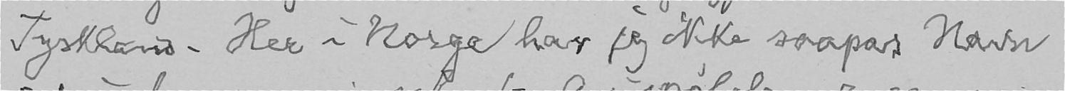Tyskland. Her i Norge har jeg ikke saapas Navn
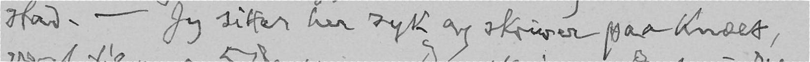stad. - Jeg sitter her syk og skriver paa Knæet,
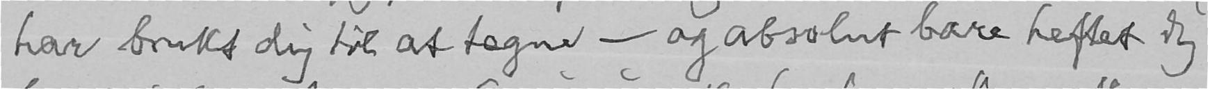har brukt dig til at tegne - og absolut bare heftet dig
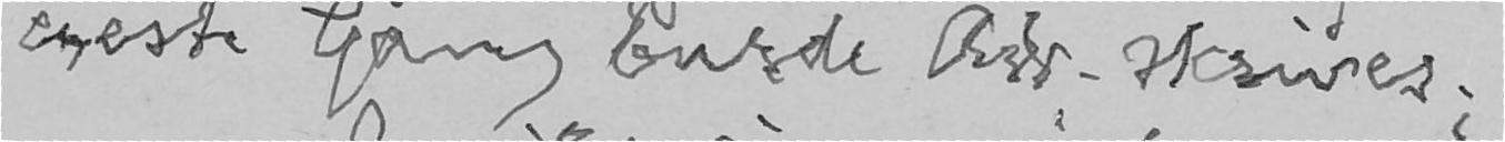eneste Gang burde Adr. skrives.
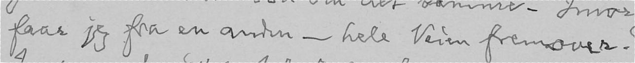faar jeg fra en anden - hele Veien fremover.
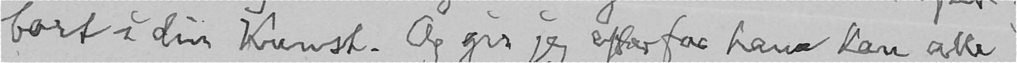bort i din Kunst. Og gir jeg efter for ham kan alle
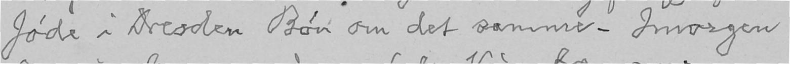Jøde i Dresden Bøn om det samme. Imorgen
